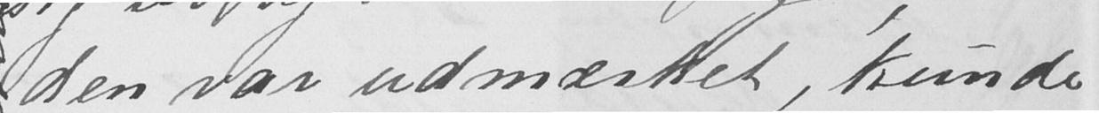den var udmærket, kunde
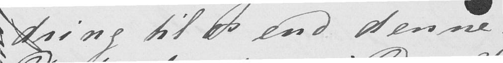dring til os end denne.
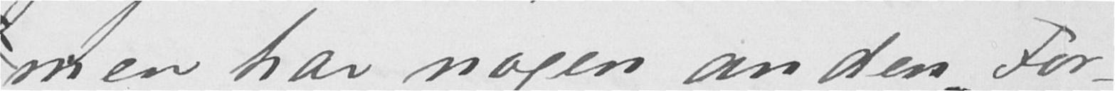men har nogen anden For-
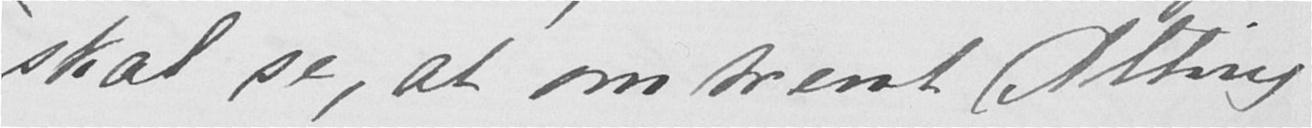skal se, at omtremt Alting
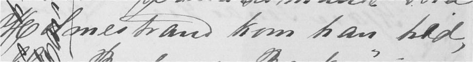Holmestrand kom han hid,
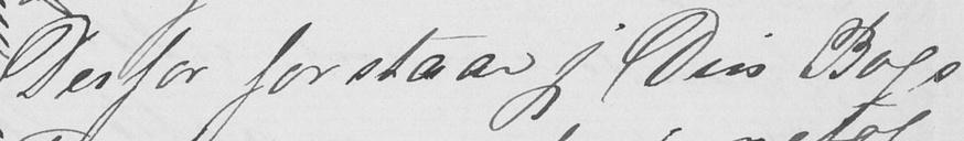Derfor forstaar jeg Din Bogs
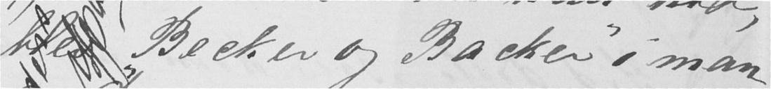blev "Backer og Backer" i man-
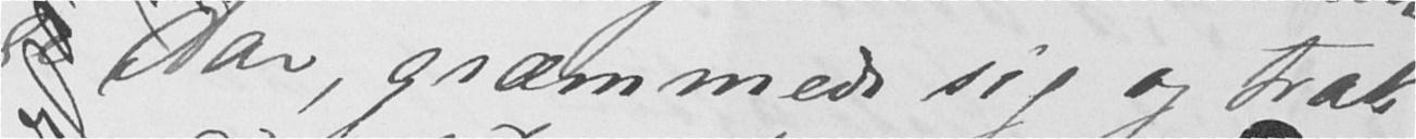ge Aar, græmmede sig og trak
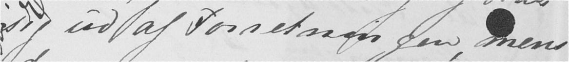sig ud af Forretningen, mens
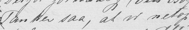Tanker saa, at vi netop
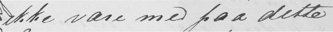ikke være med paa dette
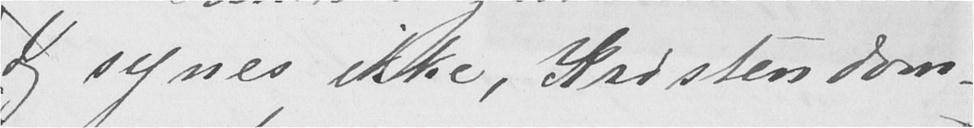Jeg synes ikke, Kristendom-
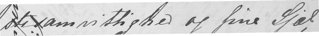stesamvittighed og fine Sjæl
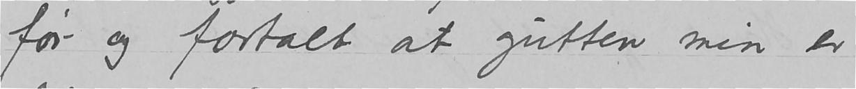før og fortalt at gutten min er
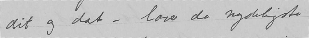dit og dat – laver de nydeligste
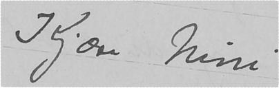Kjære Nini,
